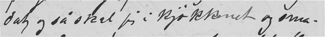dat og så skal jeg i kjøkkenet og sma-
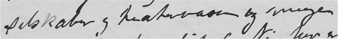selskaber og theatervæsen og megen
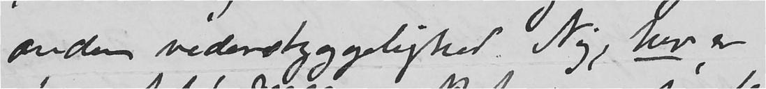anden vederstyggelighed. Nej, her er
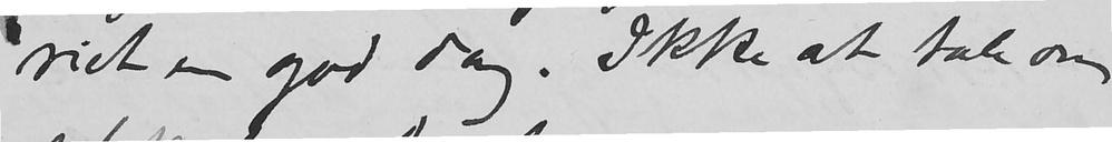riet en god dag. Ikke at tale om
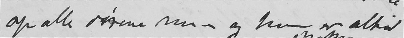op alle dørene nu - og hun er altid
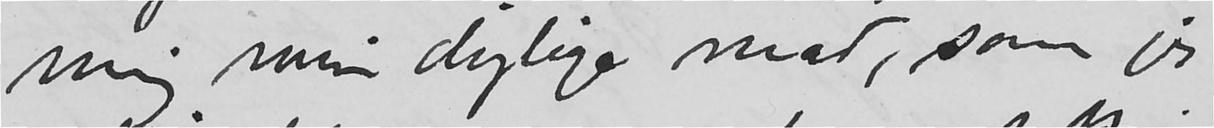mig min dejlige mad, som jeg
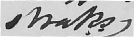straks
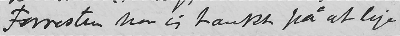Forresten har jeg tænkt på at leje
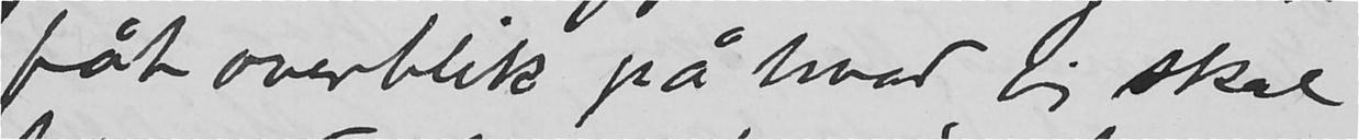fåt overblik på hvad jeg skal
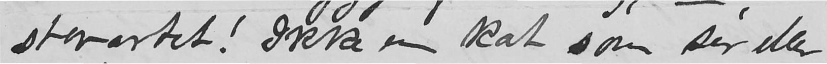storartet! Ikke en kat som ser eller
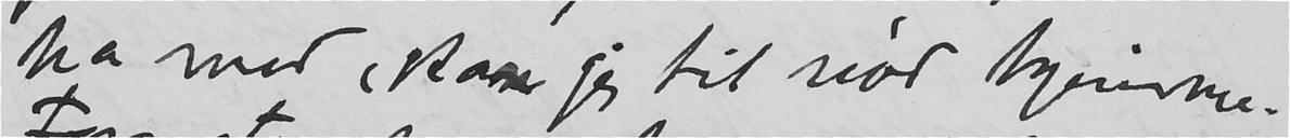ha med, kan jeg til nød hjemme.
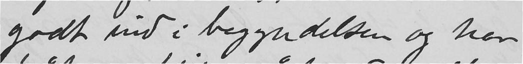godt ind i begyndelsen og har
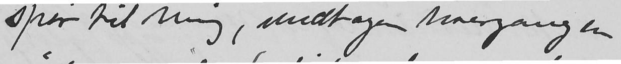spør til mig, undtagen hvergang en
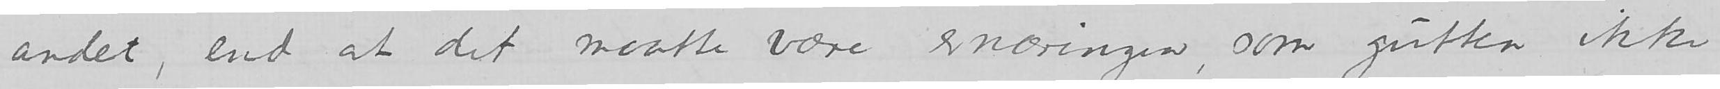andet, end at det maatte være snøringen ernæringen, som gutten ikke
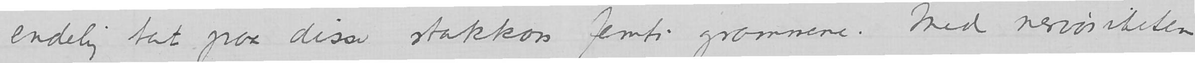endelig tat paa disse stakkars femti grammene. Med nervøsiteten
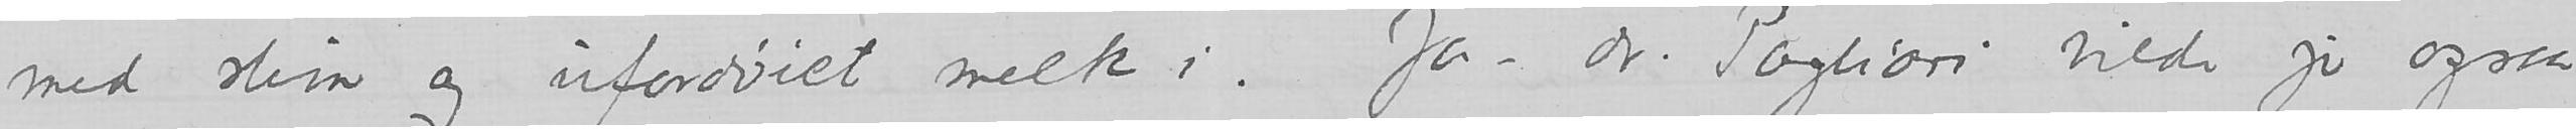med slim og ufordøiet melk i. Ja – dr. Pagliori vilde jo ogsaa
